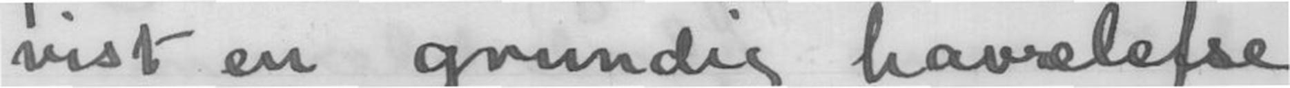vist en grundig havrelefse
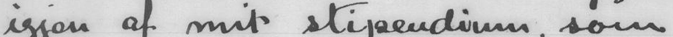igjen af mit stipendium, som
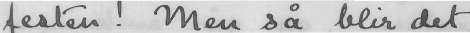festen! Men så blir det
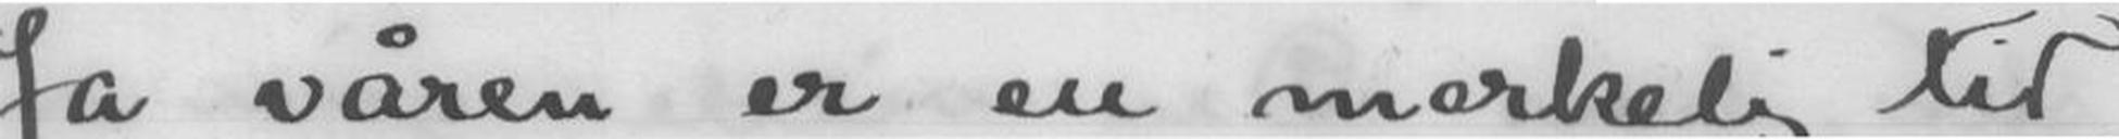Ja våren er en merkelig tid,
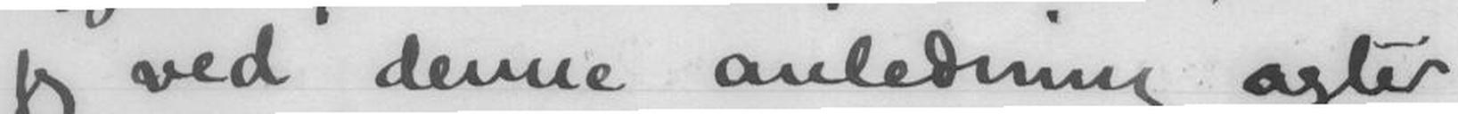jeg ved denne anledning agter
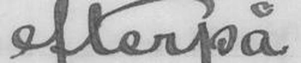efterpå
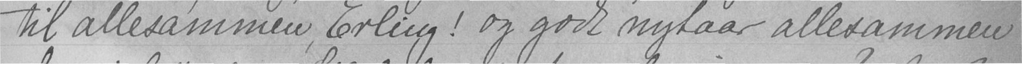til allesammen, Erling! og godt nytaar allesammen
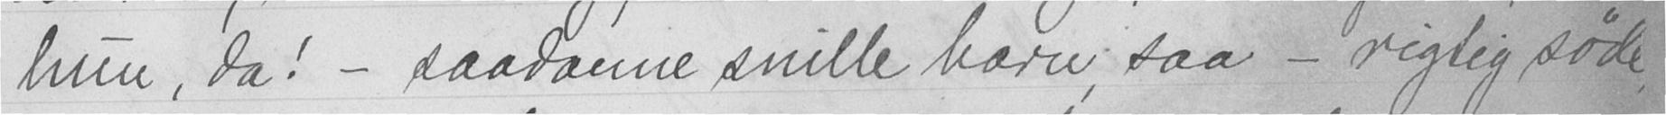hun, da! - saadanne snille barn, saa - rigtig søde
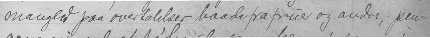mangled paa overtalelser baade fra fruer og andre, - pen-
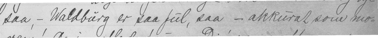saa, - Waldburg er saa ful, saa - akkurat som mo-
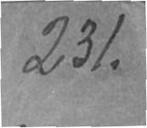231.
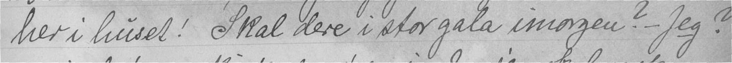her i huset! Skal dere i stor gala imorgen? - Jeg?
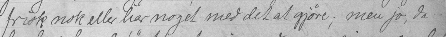frisk nok eller har noget med det at gjøre; men jo, da -
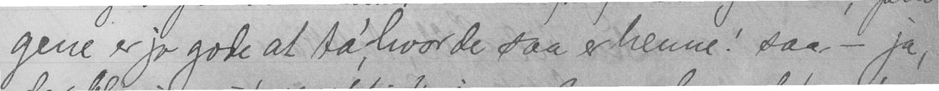gene er jo gode at ta', hvorde saa er henne! saa - ja,
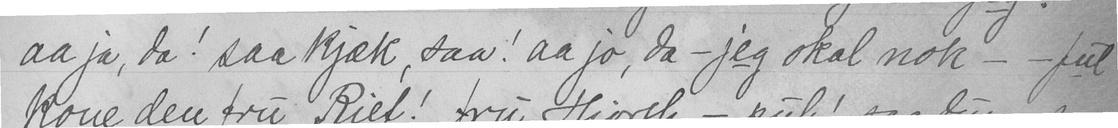aa ja, da! saa kjæk, saa! aa jo, da - jeg skal nok - - ful
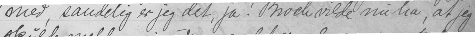med, sandelig er jeg det, ja! Broch vilde nu ha, at jeg
